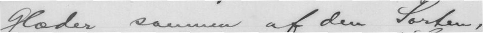Glæder sammen af den Sorten,
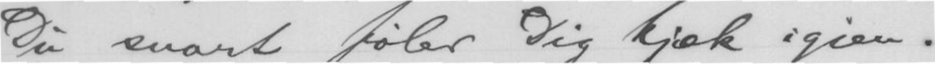Du snart føler Dig kjæk igjen.
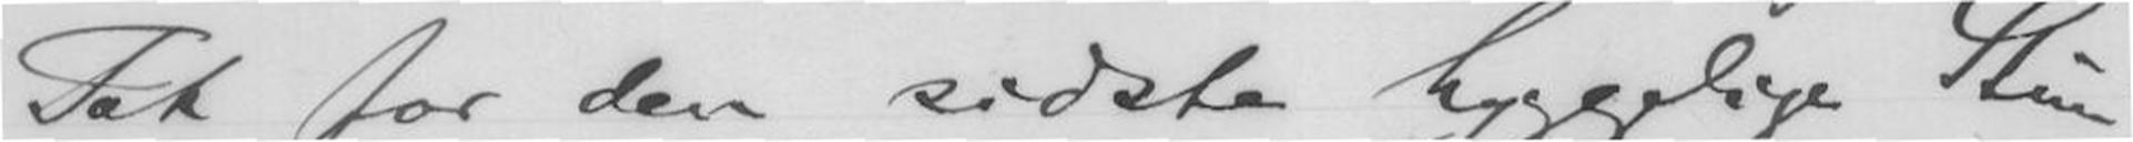Tak for den sidste hyggelige Stun-
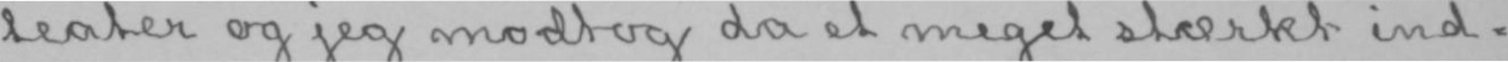teater og jeg modtog da et meget stærkt ind-
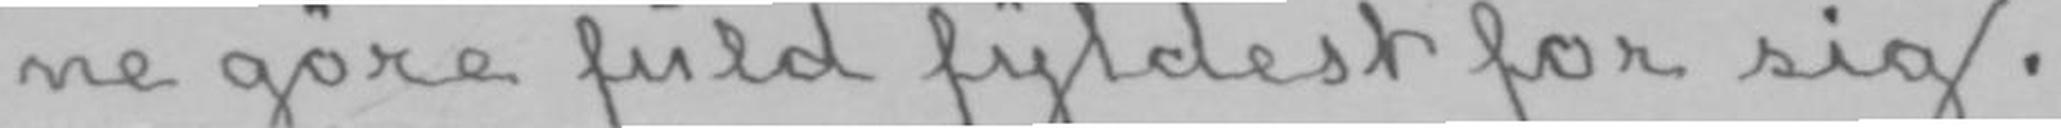ne gøre fuld fyldest for sig.
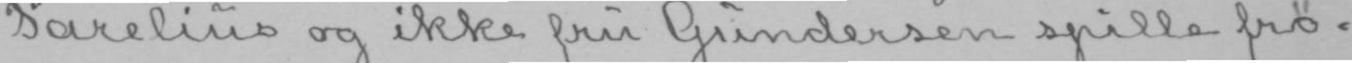Parelius og ikke fru Gundersen spille frø-
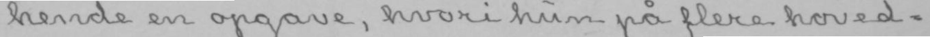hende en opgave, hvori hun på flere hoved-
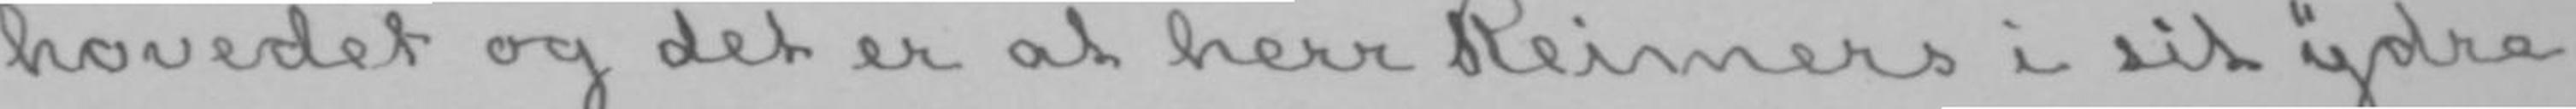hovedet og det er at herr Reimers i sit ydre
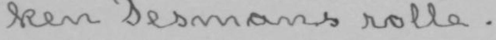ken Tesmans rolle.
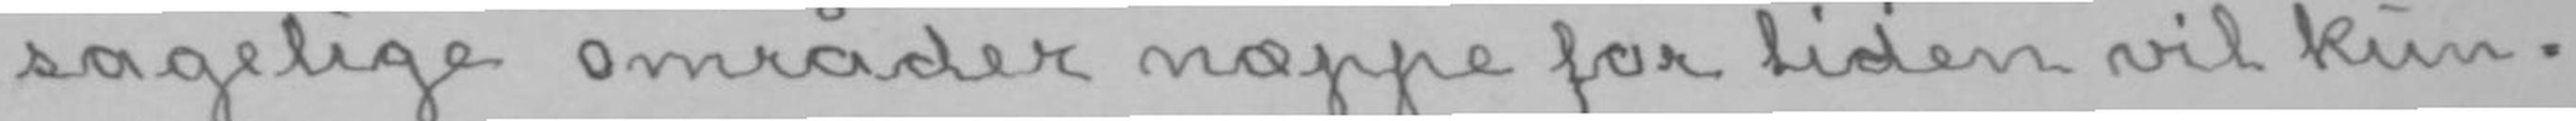sagelige områder næppe for tiden vil kun-
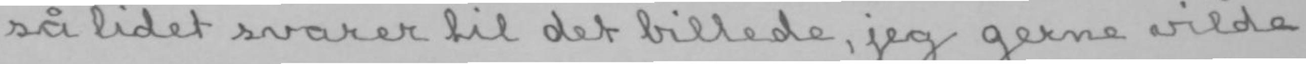så lidet svarer til det billede, jeg gerne vilde
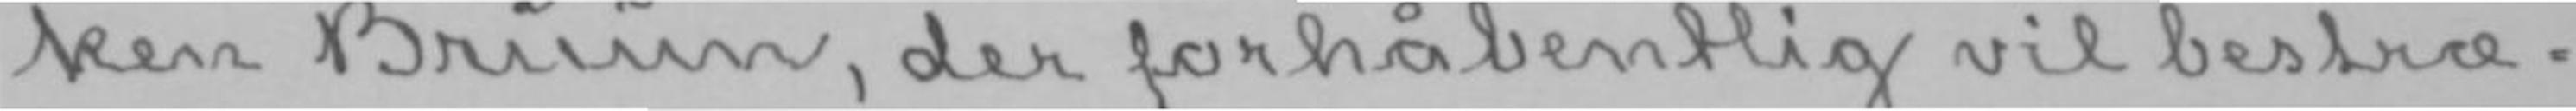ken Bruun, der forhåbentlig vil bestræ-
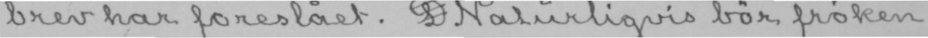brev har foreslået. D Naturligvis bør frøken
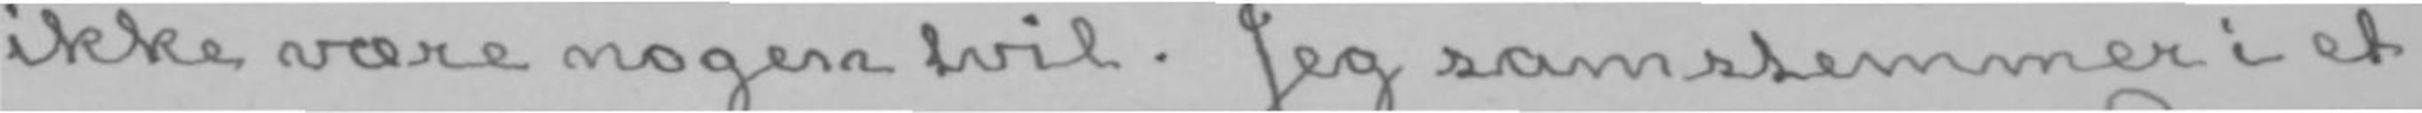ikke være nogen tvil. Jeg samstemmer i et
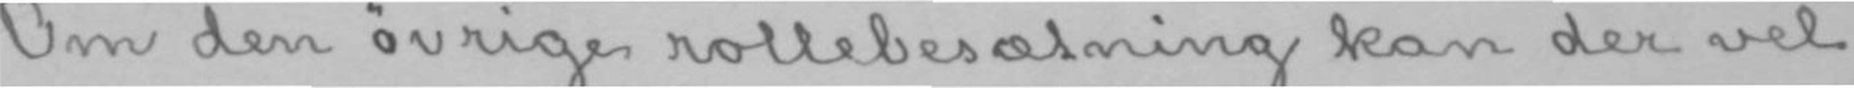Om den øvrige rollebesætning kan der vel
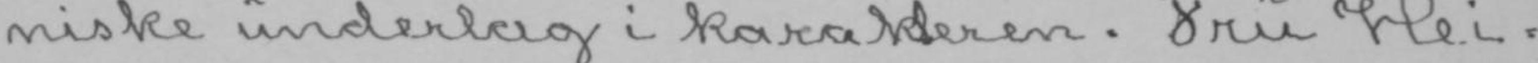niske underlag i karakteren. Fru Hei-
